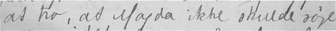at tro, at Magda ikke skulde søge
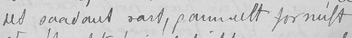det saadant rart, gammelt fornuft
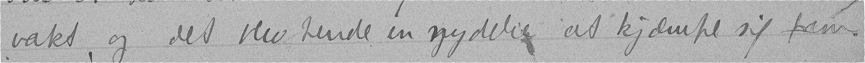vakt, og det blev hende en nydelse at kjæmpe sig frem-
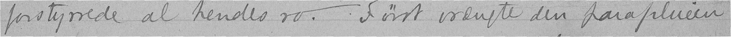forstyrrede al hendes ro. Først vrængte den parapluien
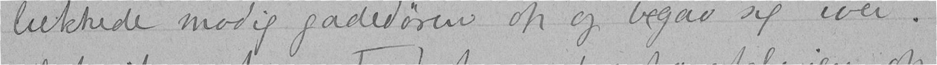lukkede modig gadedøren op og begav sig ivei.
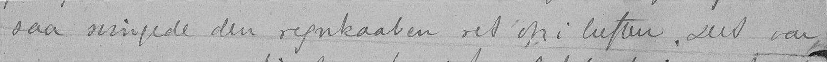saa svingede den regnkaaben ret op i luften. Det var
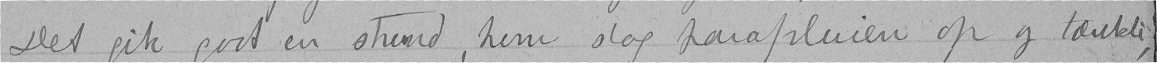Det gik godt en stund, hun slog parapluien op og tænkte,
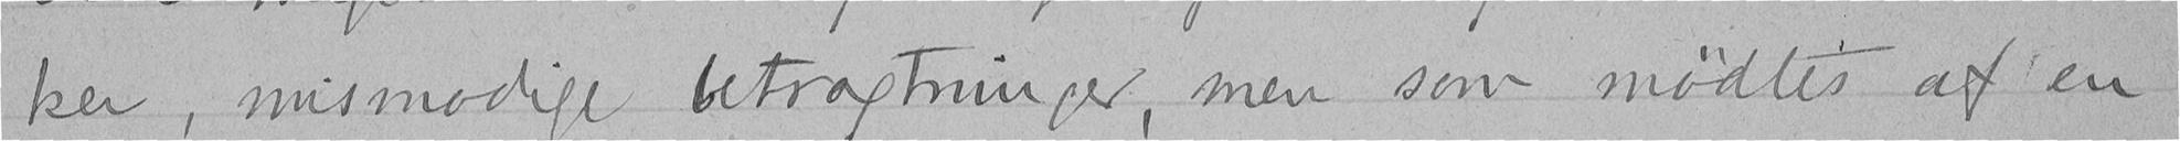ker, mismodige betragtninger, men som mødtes af en
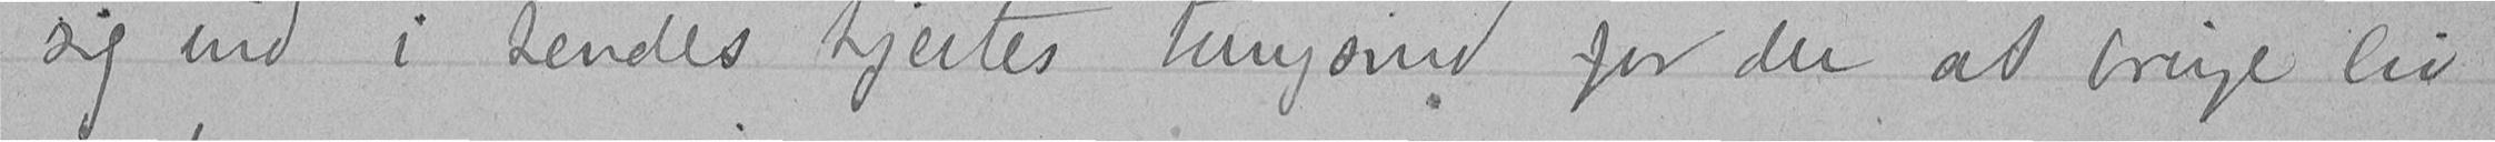sig ind i hendes hjertes tungsind for der at bringe liv
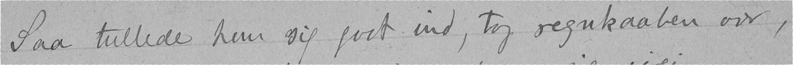Saa tullede hun sig godt ind, tog regnkaaben over,
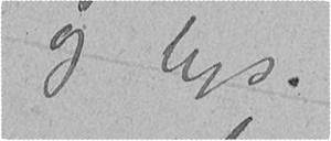og lys.
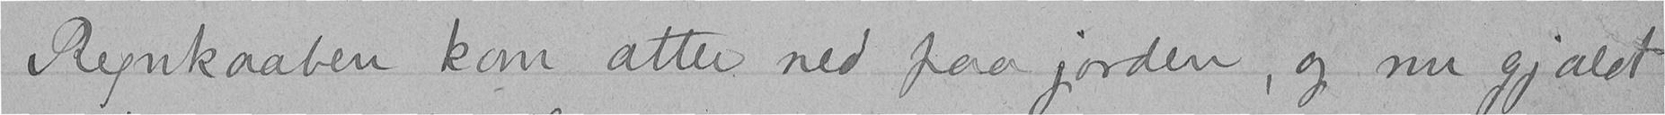Regnkaaben kom atter ned paa jorden, og nu gjaldt
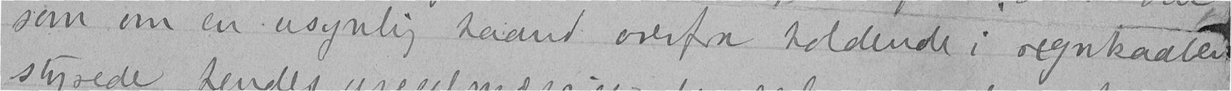som om en usynlig haand overfra holdende i regnkaaben
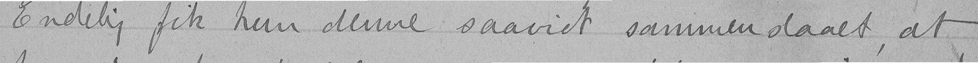Endelig fik hun denne saavidt sammenslaaet, at
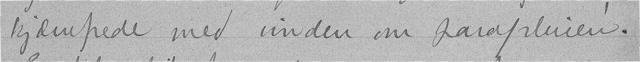kjæmpede med vinden om parapluien.
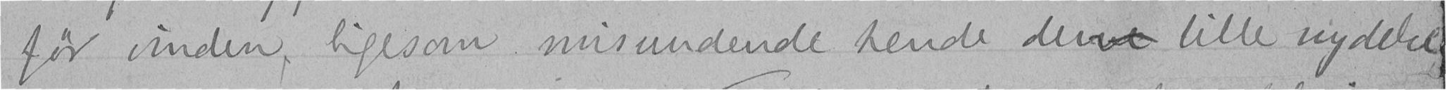før vinden, ligesom misundende hende denne lille nydelse
